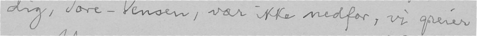dig, Tore-Vensen, vær ikke nedfor, vi greier
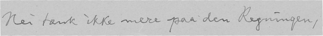Nei tænk ikke mere paa den Regningen,
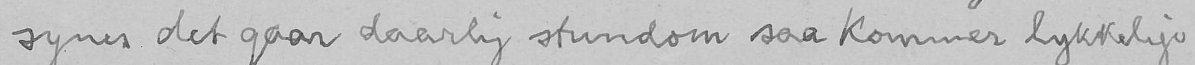synes det gaar daarlig stundom saa kommer lykkelige
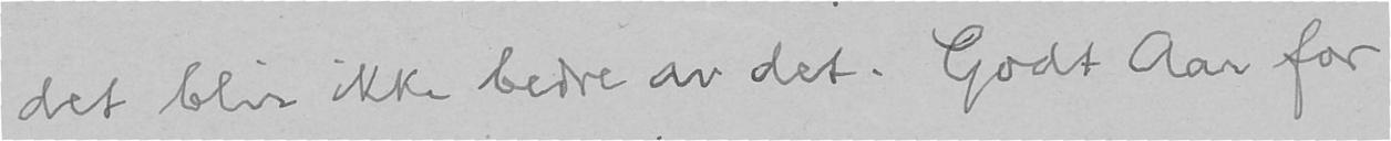det blir ikke bedre av det. Godt Aar for
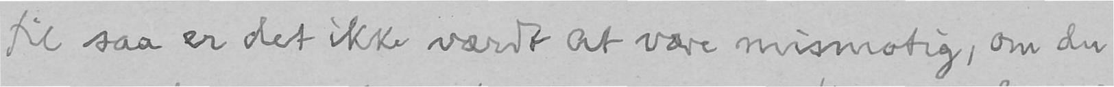til saa er det ikke værdt at være mismotig, om du
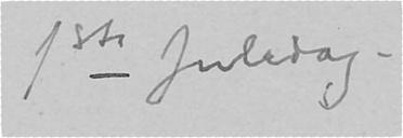1ste Juledag.
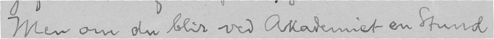Men om du blir ved Akademiet en Stund
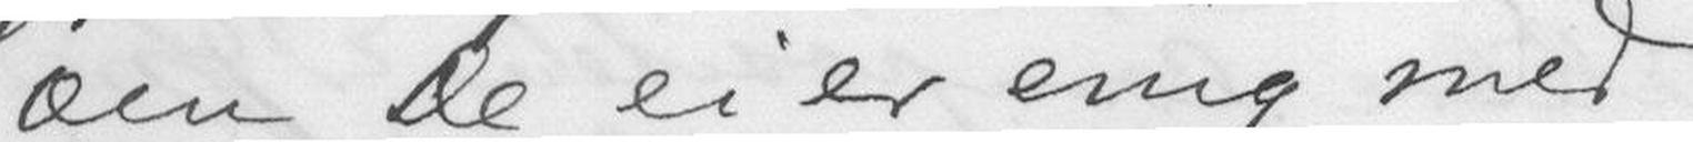om De ei er enig med
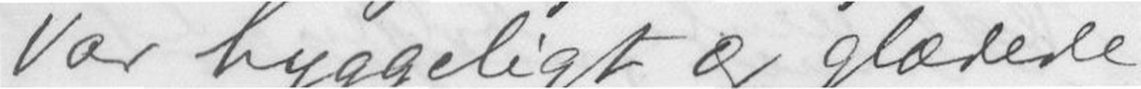var hyggeligt og glædede
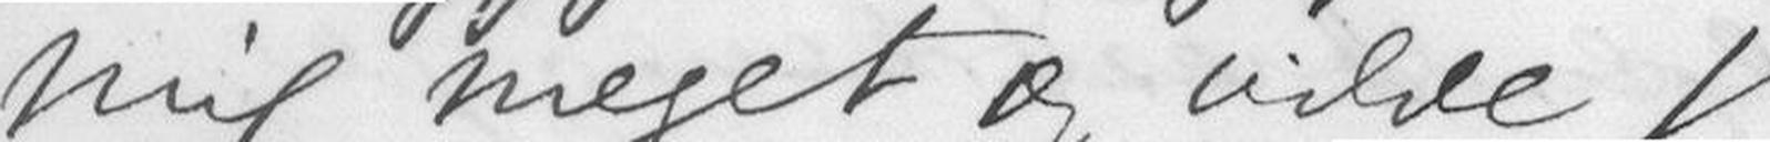mig meget og vilde jeg
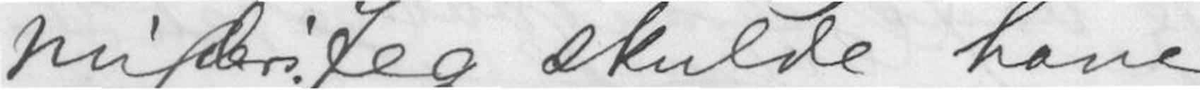mig deri. Jeg skulde have
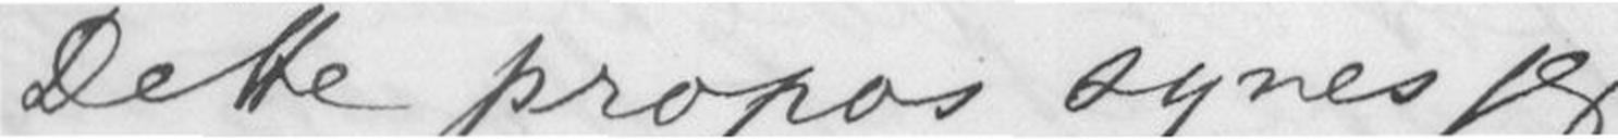Dette propos synes jeg
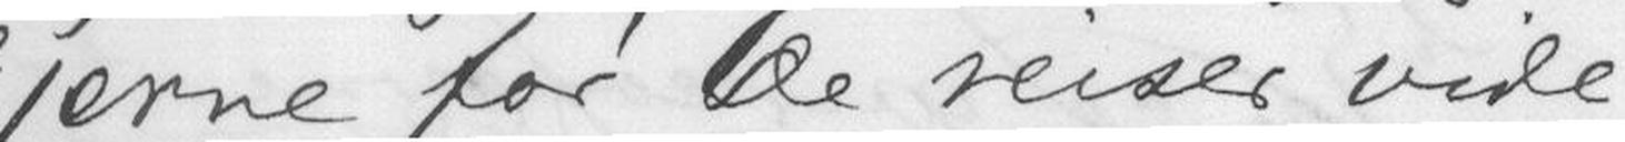gjerne før De reiser vide
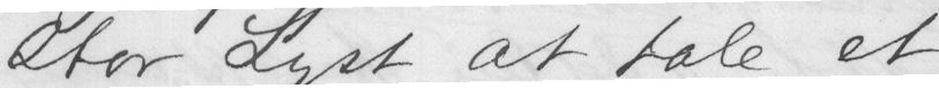stor Lyst at tale et
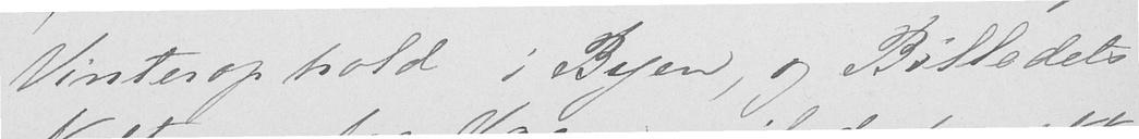Vinterophold i Byen, og Billedets
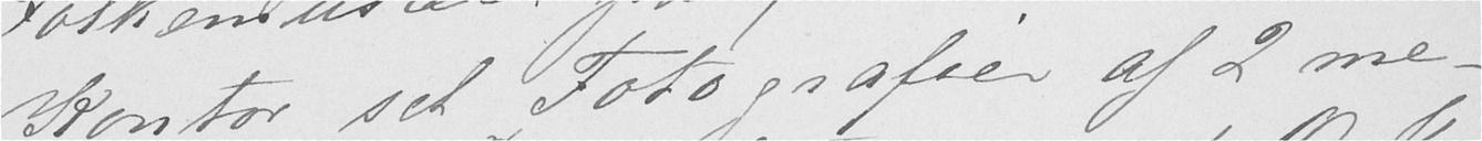Kontor set Fotografier af 2 me-
